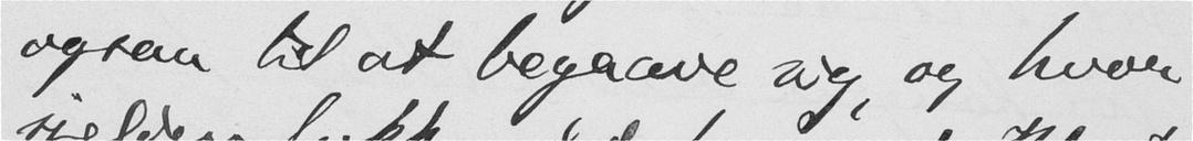ogsaa til at begrave sig, og hvor
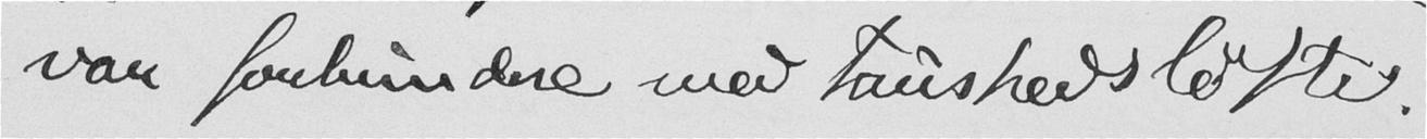var forbundne med taushedsløftet.
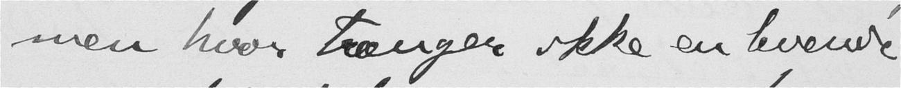men hvor trænger ikke en levende
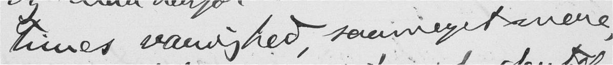times varighed, saameget mere
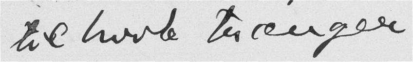til hvile trænger
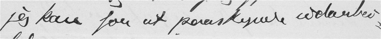jeg kan for at paaskynde udarbei-
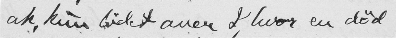ak, kun lidet aner I, hvor en død
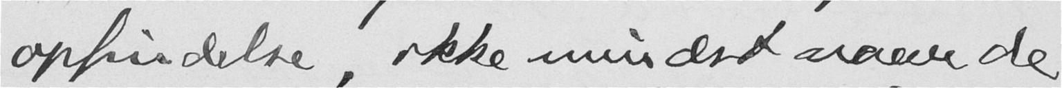opfindelse, ikke mindst naar de
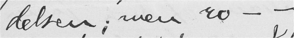delsen; men ro - -
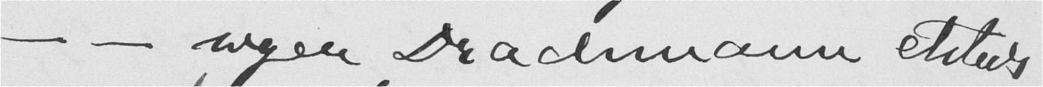- - siger Drachmann etsteds;
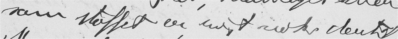som stoffet er rigt nok dertil.
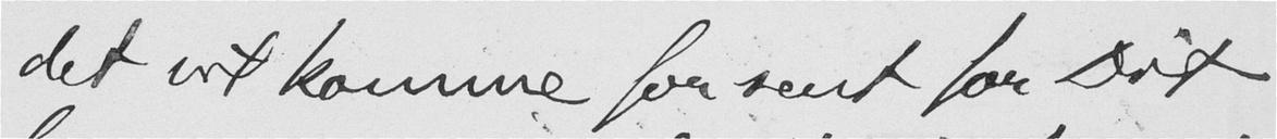det vil komme for sent for Dit
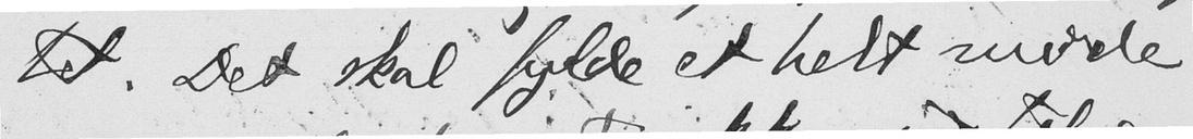tet. Det skal fylde et helt møde
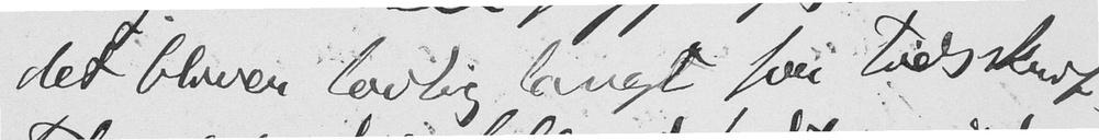det bliver lovlig langt for tidsskrif-
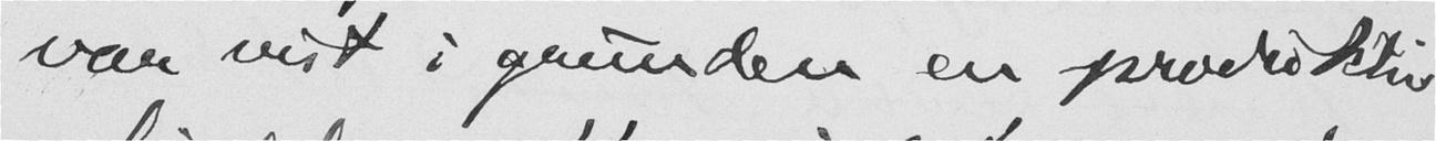var vist i grunden en produktiv
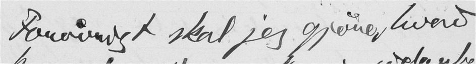Forøvrigt skal jeg gjøre, hvad
